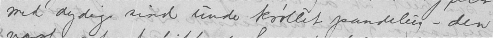med dydige sind under krøllet pandelug - den
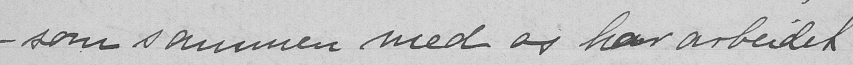- som sammen med os har arbeidet
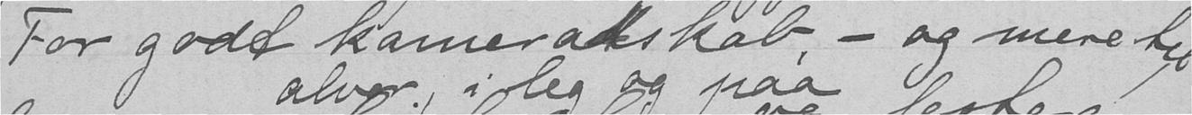For godt kameratskab, - og mere til
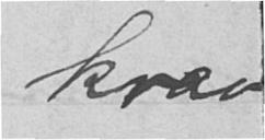krav
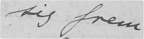sig frem
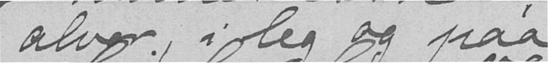alvor, i leg og paa
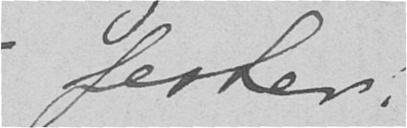fester!
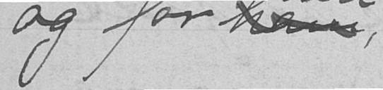og for ham,
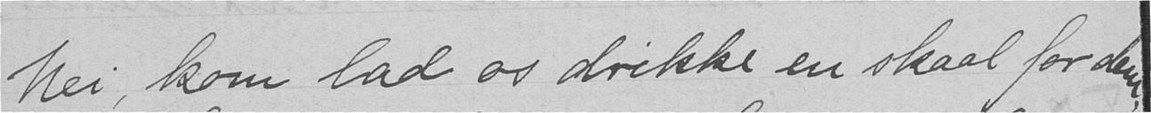Nei, kom lad os drikke en skaal for dem
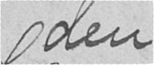den
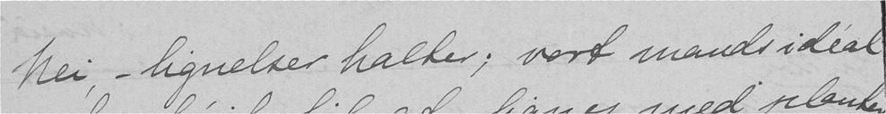Nei, - lignelser halter; vort mandsideal
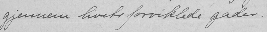gjennem livets forviklede gader.
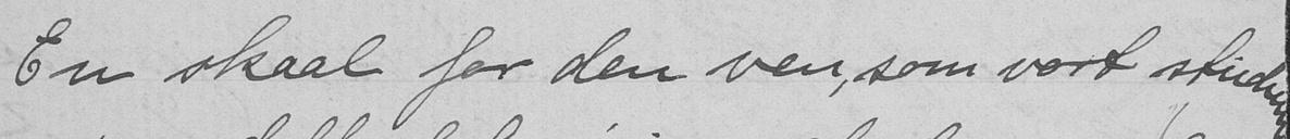En skaal for den ven, som vort studium
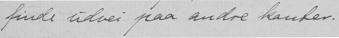finde udvei paa andre kanter.
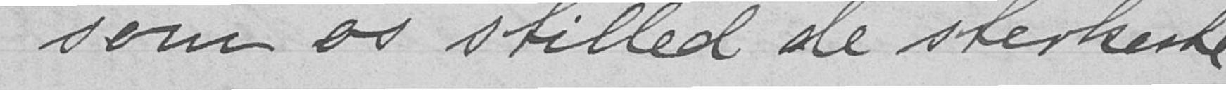som os stilled de sterkeste
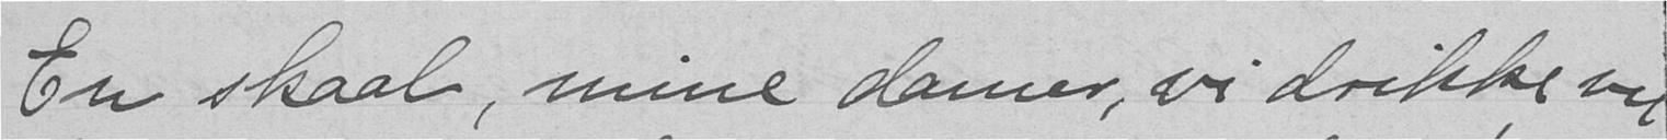En skaal, mine damer, vi drikke vil
